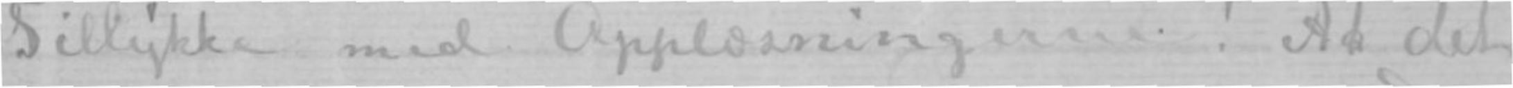Tillykke med Opplæsningerne! At det
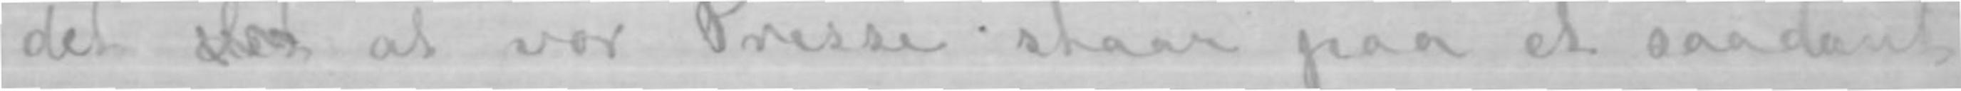det det at vor Presse staar paa et saadant
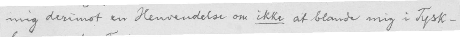mig derimot en Henvendelse om ikke at blande mig i Tysk-
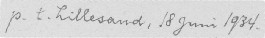p. t. Lillesand, 18 Juni 1934.
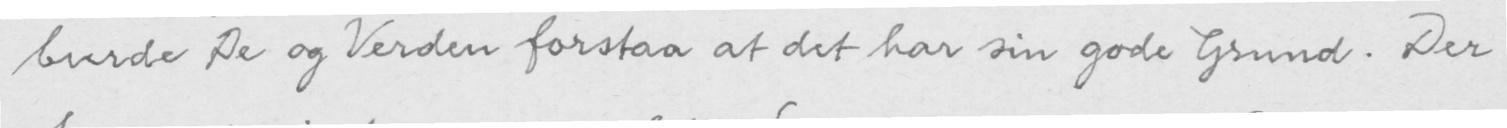burde De og Verden forstaa at det har sin gode Grund. Der
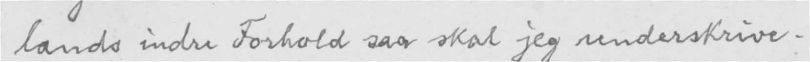lands indre Forhold saa skal jeg underskrive.
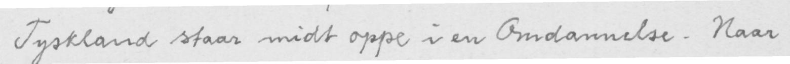Tyskland staar midt oppe i en Omdannelse. Naar
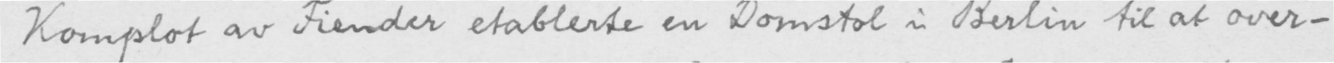Komplot av Fiender etablerte en Domstol i Berlin til at over-
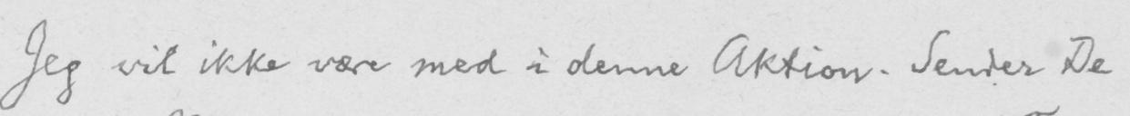Jeg vil ikke være med i denne Aktion. Sender De
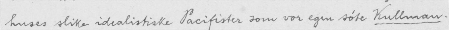huses slike idealistiske Pacifister som vor egen søte Kullman.
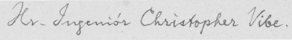Hr. Ingeniør Christopher Vibe.
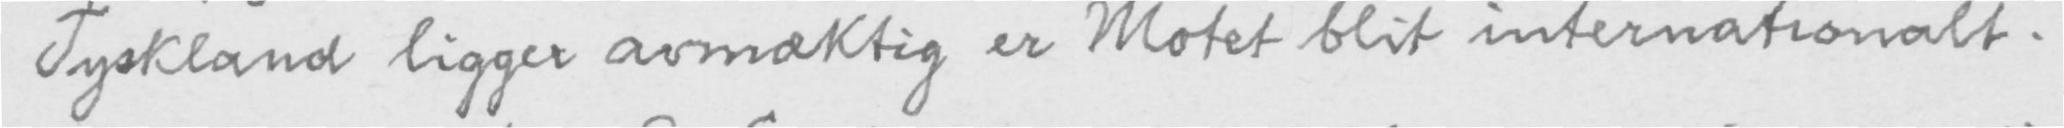Tyskland ligger avmæktig er Motet blit internationalt.
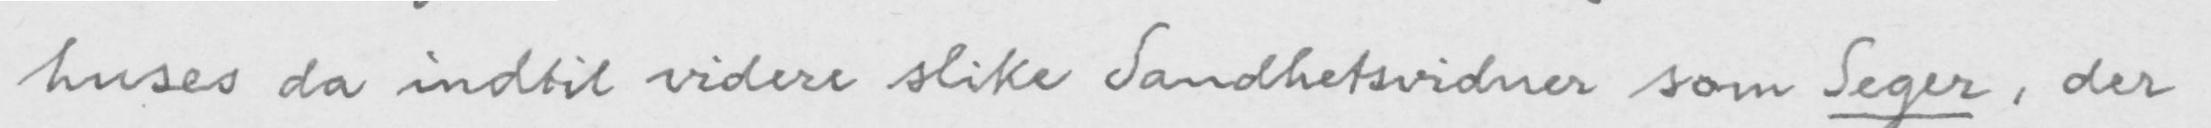huses da indtil videre slike Sandhetsvidner som Seger, der
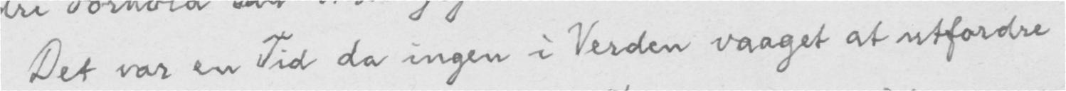Det var en Tid da ingen i Verden vaaget at utfordre
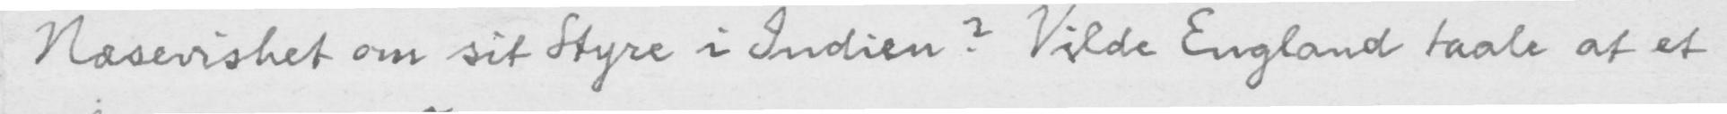Næsevishet om sit Styre i Indien? Vilde England taale at et
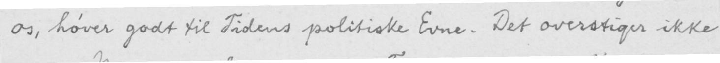os, høver godt til Tidens politiske Evne. Det overstiger ikke
